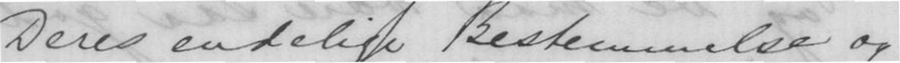Deres endelige Bestemmelse og
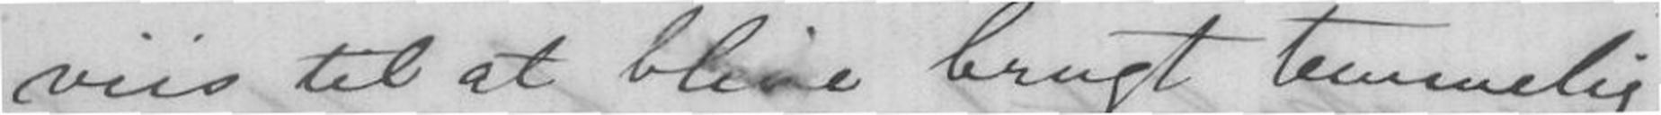viis til at blive temmelig
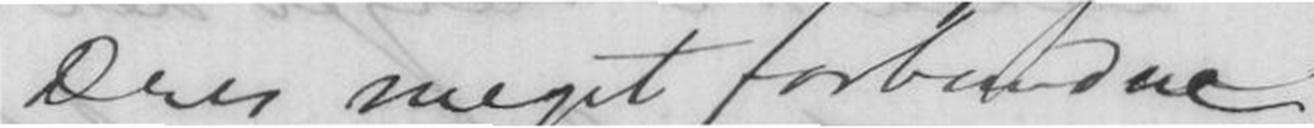Deres meget forbundne
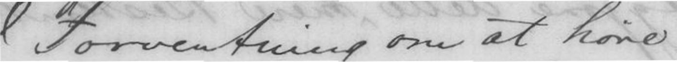I Forventning om at høre
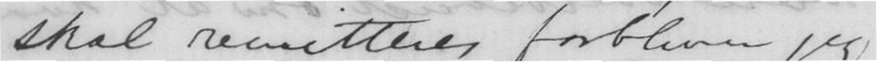skal remitteres forbliver jeg
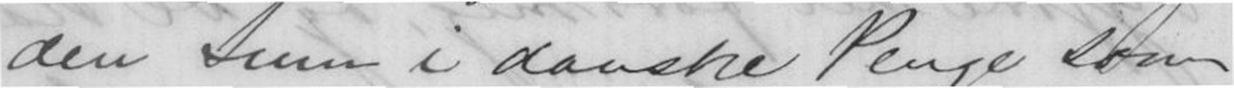den Sum i danske Penge som
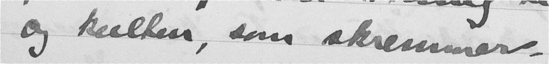og kultur, som skremmer.
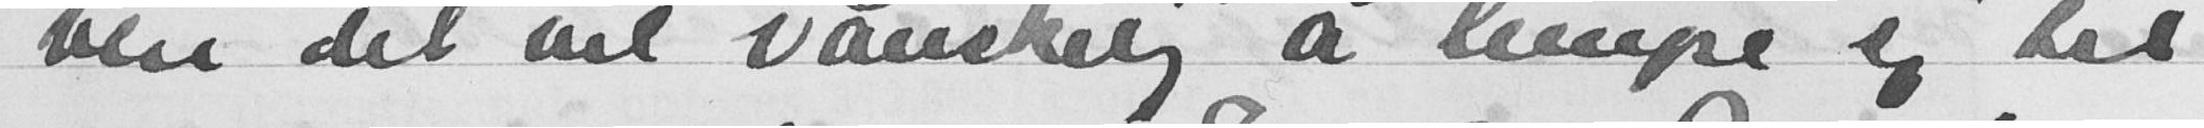blir det vel vanskelig å lempe sig til
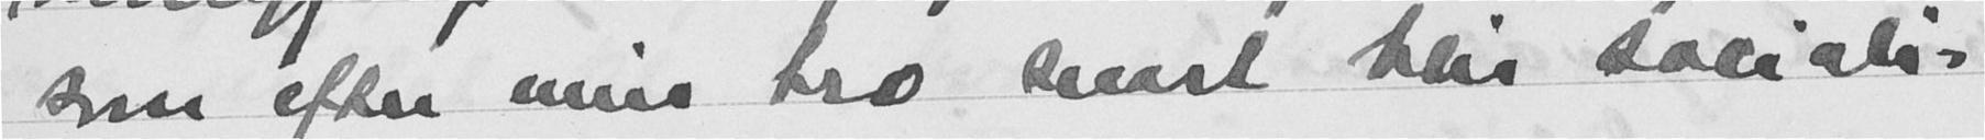som efter min tro snart blir sociali-
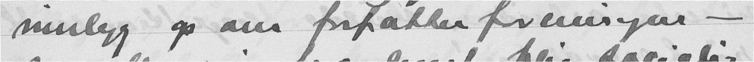innlegg op om forfatterforeningen -
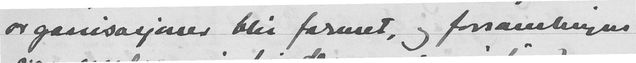organisasjoner blir formet, og forsamlingen
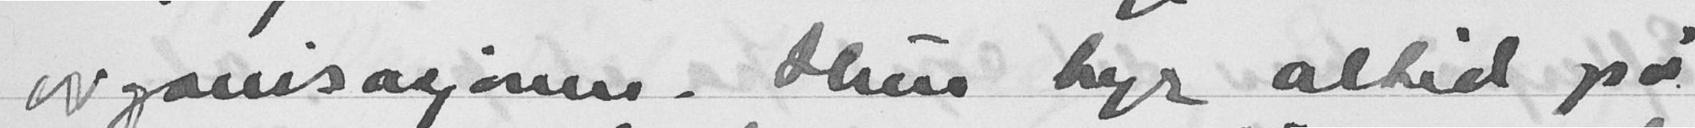organisasjonen. Hun byr altid på
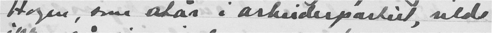Hagen, som står i arbeiderpartiet, vilde
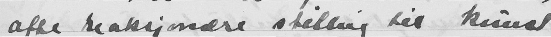ofte reaksjonære stilling til kunst
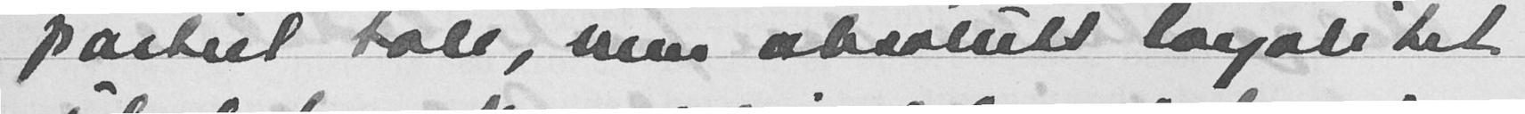partiet tåle, men absolutt loyalitet
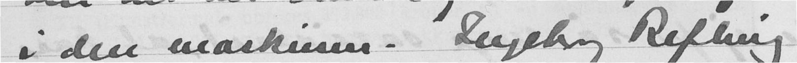i den maskinen. Ingeborg Refling
